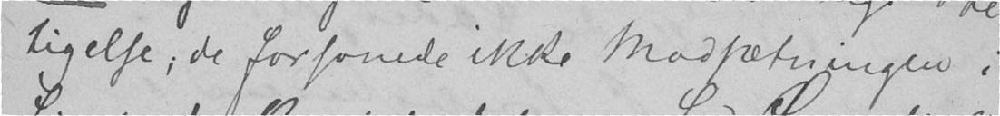tigelse; de forsonede ikke Modsætningen i
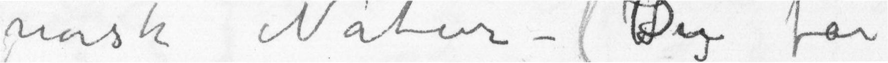norsk Natur (Du får
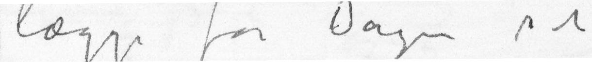lægge for Dagen sit
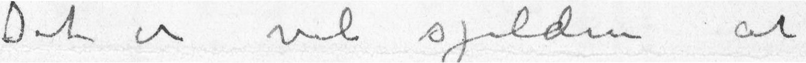Det er vel sjelden at
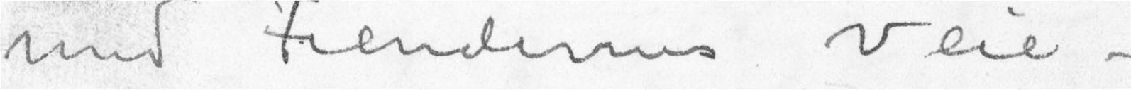med Fiendens Veie –
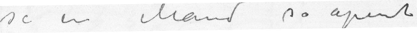se en Mand så åpent
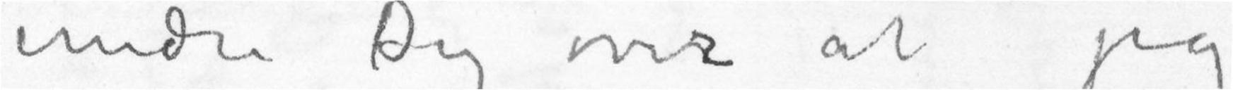undre Dig over at jeg
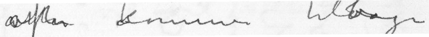atterofte kommer tilbage
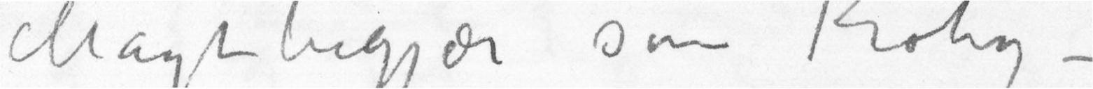Magtbegjær som Krogh –
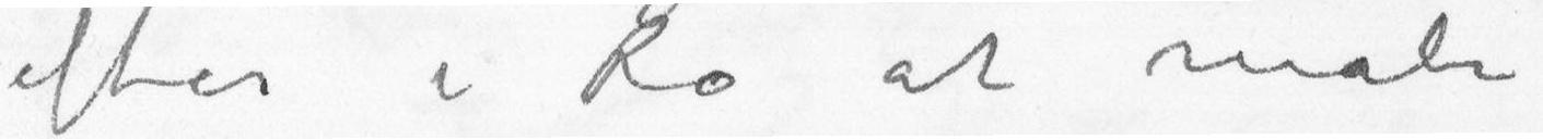efter i Ro at male
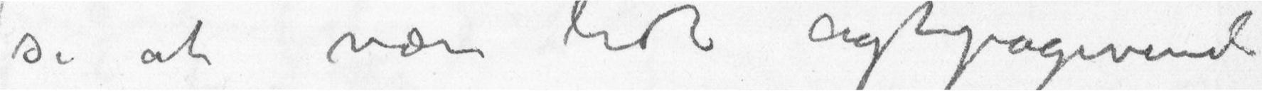se at være lidt agtpågivende
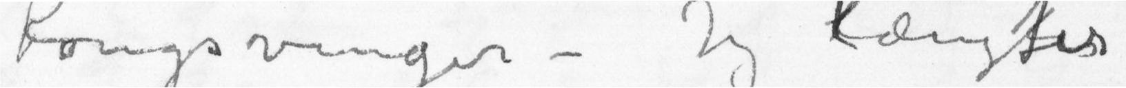Kongsvinger – Jeg længter
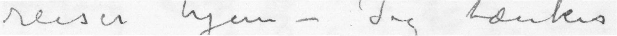reiser hjem – Jeg tænker
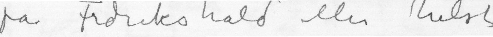på Fredrikshald eller helst
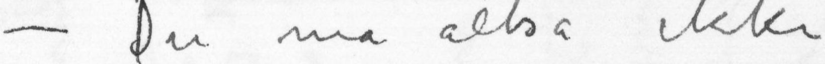– Du må altså ikke
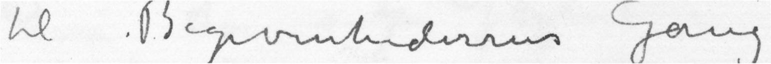til Begivenhedernes Gang
